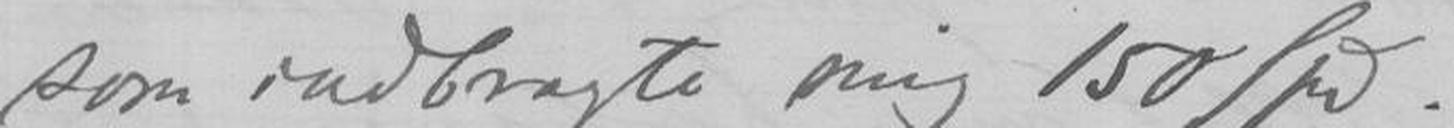som indbragte mig 150 Spd.
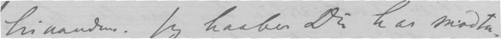hinanden. Jeg haaber Du har mod-
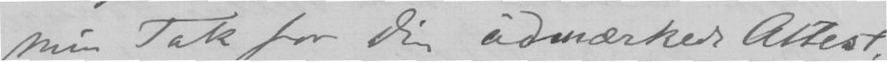min Tak for Din udmærkede Attest,
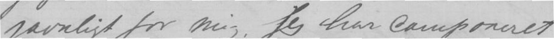gavnlig for mig. Jeg har componeret
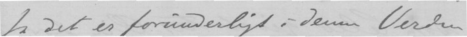Ja det er forunderligt i denne Verden,
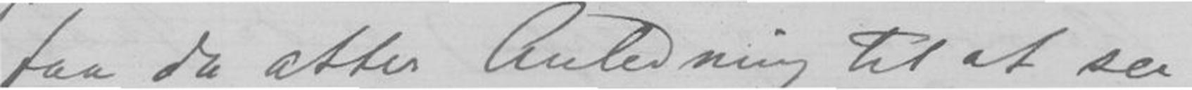faa da atter Andledning til at se
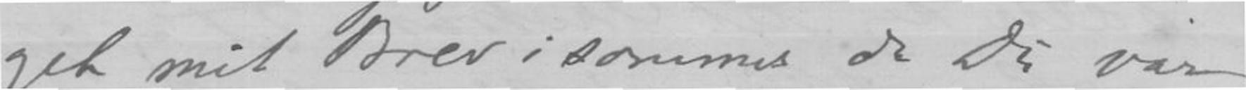taget mit Brev i sommer da Du var
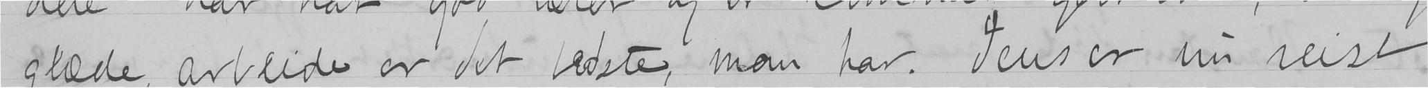glæde, arbeide er det bedste, man har. Jens er nu reist
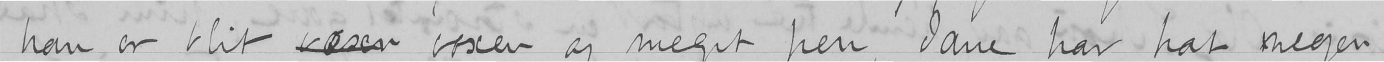han er blit vosen voxen og meget pen, Jane har hat megen
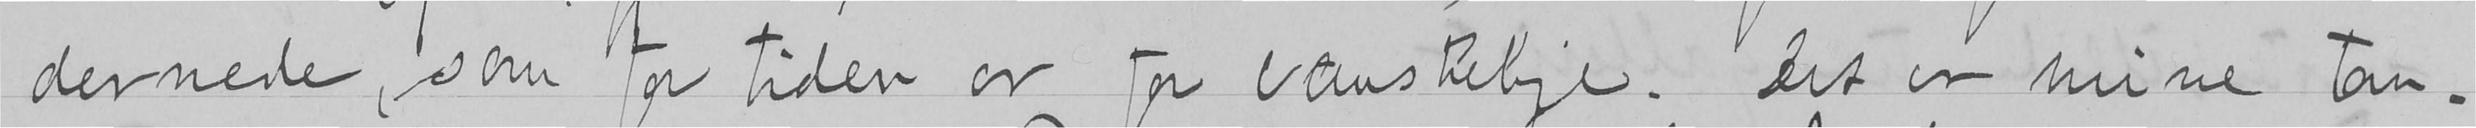dernede, som for tiden er for vanskelige. Det er mine tan-
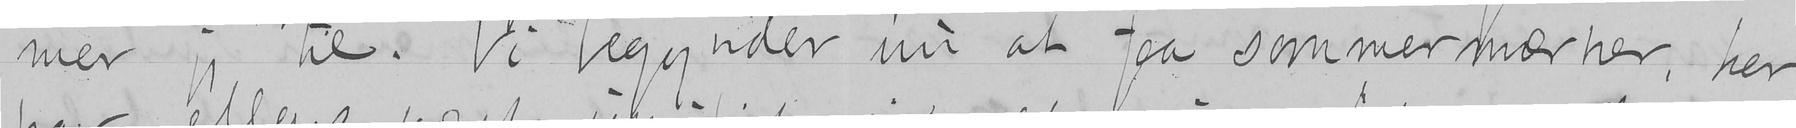mer jeg til. Vi begynder nu at faa sommermærker, her
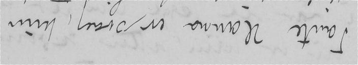Tante Hanne er svag, hun
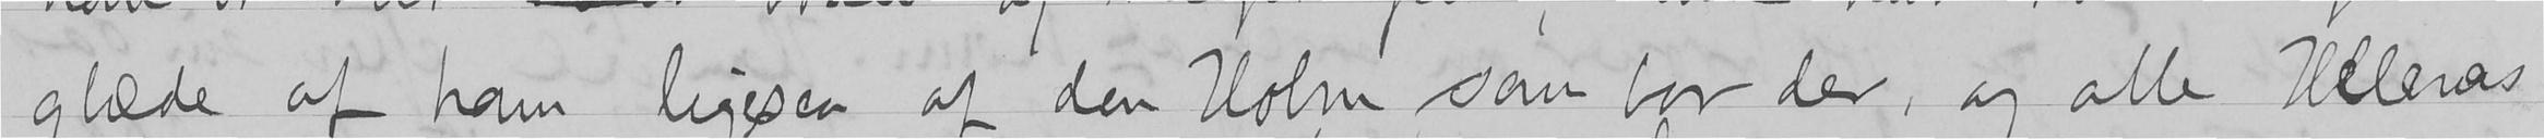glæde af ham ligesaa af den Holm som bor der, og alle Helenes
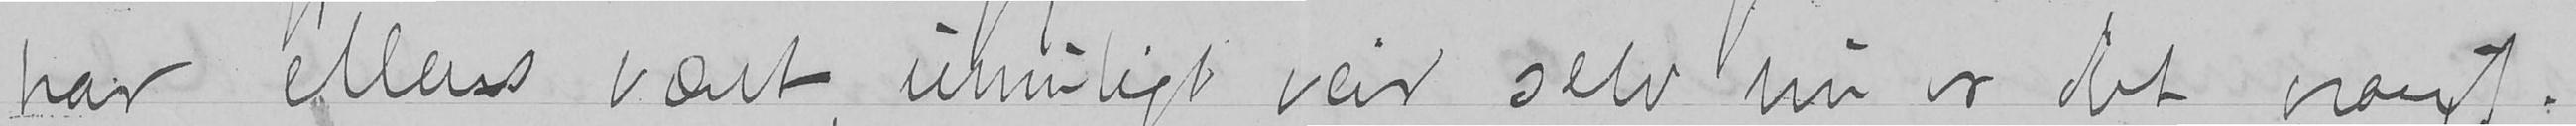har ellers været umuligt veir selv nu er det vrangt.
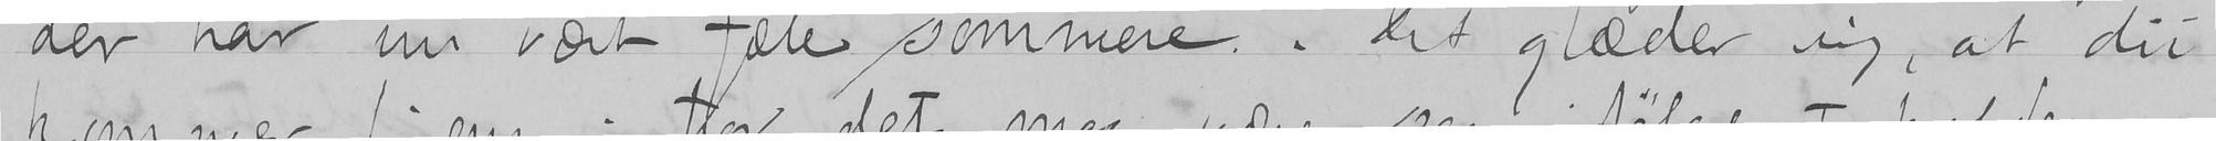der har nu vært fæle sommere. Det glæder mig, at du
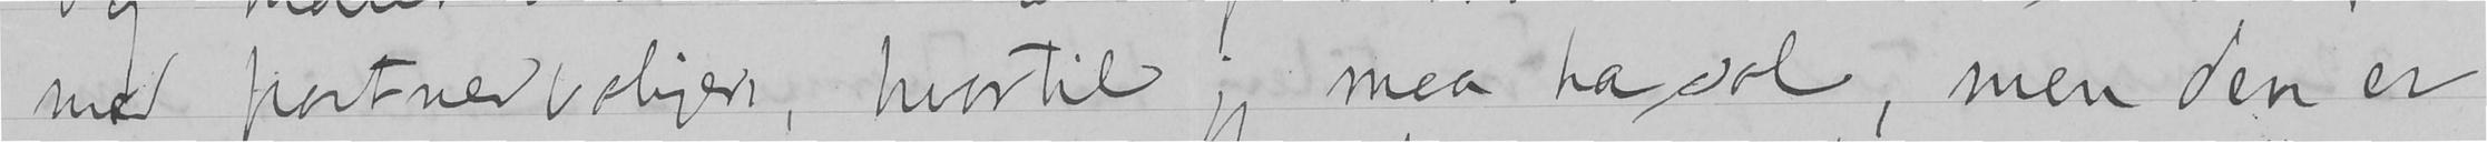med portnerboliger, hvortil jeg maa ha sol, men den er
